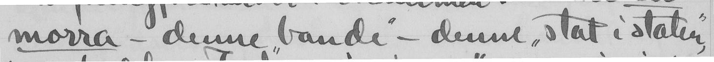morra - denne "bande" - denne "stat i staten",
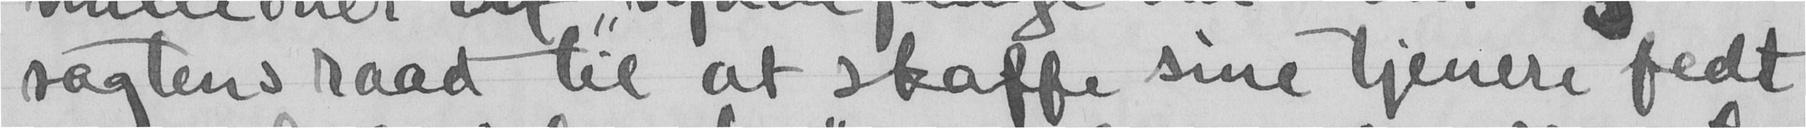sagtens raad til at skaffe sine tjenere fedt
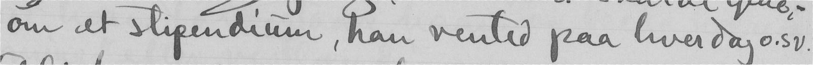om et stipendium, han vented paa hver dag o.s.v.
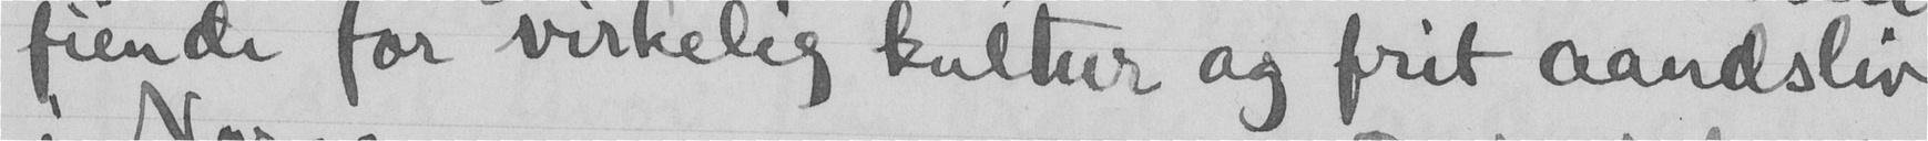fiende for virkelig kultur og frit aandsliv
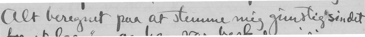Alt beregnet paa at stemme mig gunstig sindet
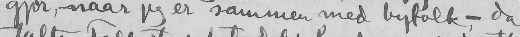gjør, aar jeg er sammen med byfolk - da
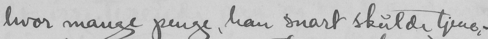hvor mange penge, han snart skulde tjene, -
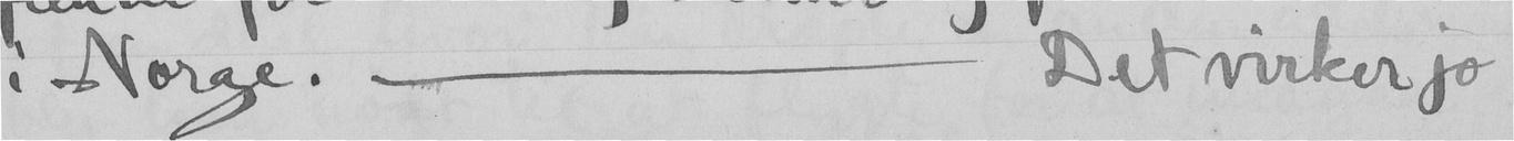i Norge! - Det virker jo
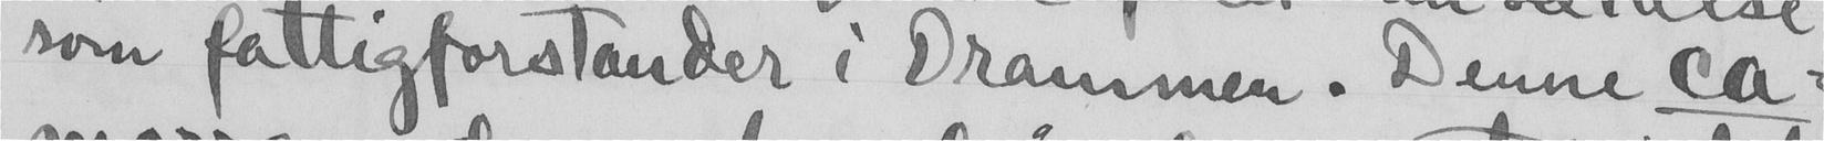som fattigforstander i Drammen. Denne ca-
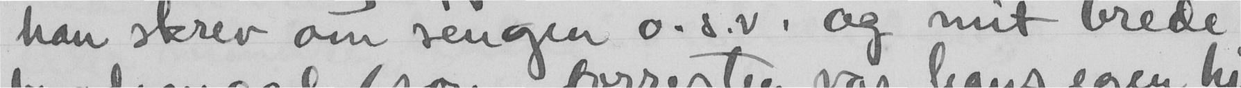han skrev om sengen o.s.v. og mit brede
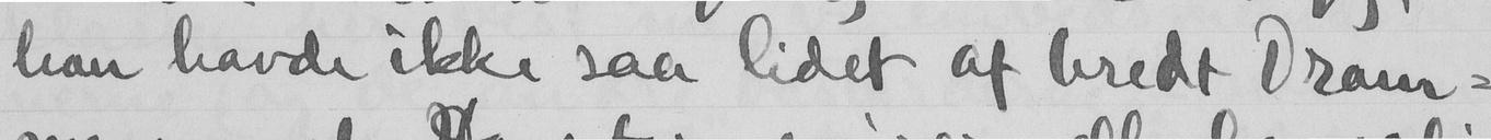han havde ikke saa lidet af bredt Dram-
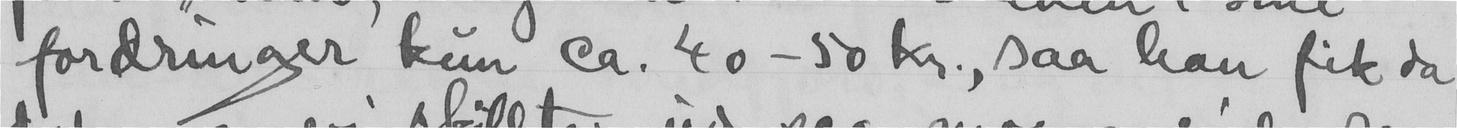fordringer kun ca. 40-50 kr, saa han fik da
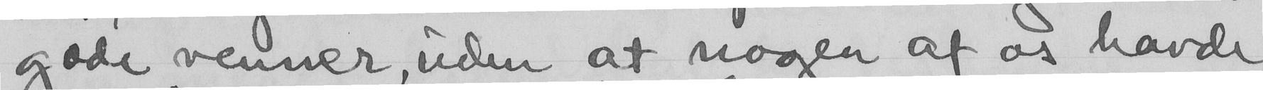gode venner, uden at nogen af os havde
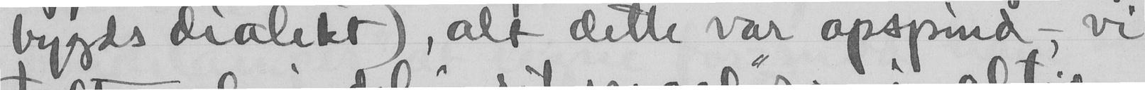bygds dialekt), alt dette var opspind- vi
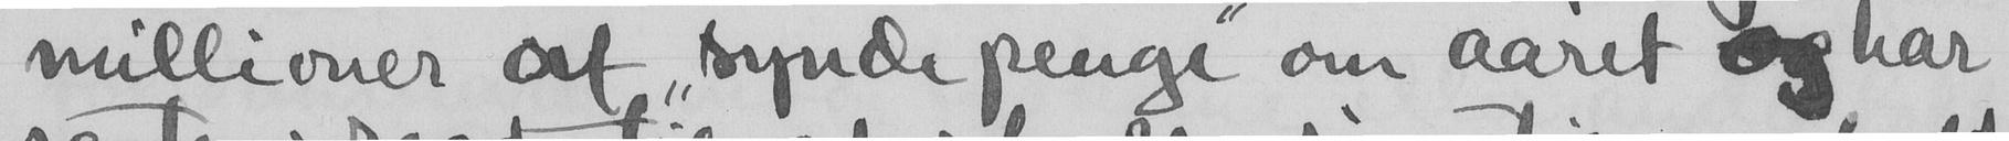millioner af "synde penge" om aaret og har
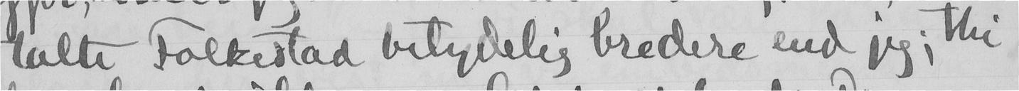talte Folkestad betydelig bredere end jeg; thi
